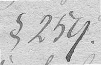§ 259.
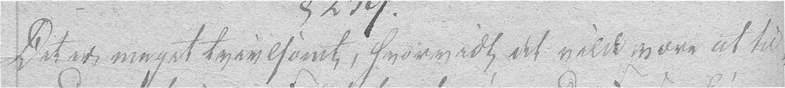Det er meget tvivlsomt, hvorvidt det vilde være at til-
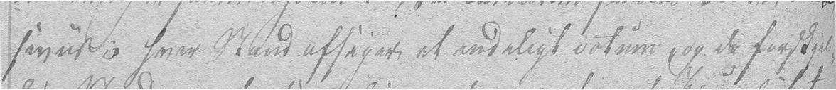sevis og hver Mand afsiger et endeligt votum, og de forskjel-
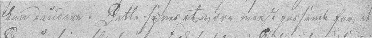kan decidere. Dette synes at være meest passende for et
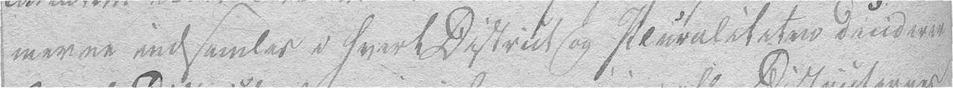merne indsamles i hver District og Pluraliteten deciderer
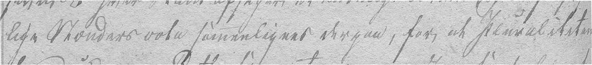lige Stænders vota sammenlignes derpaa, for at Pluraliteten
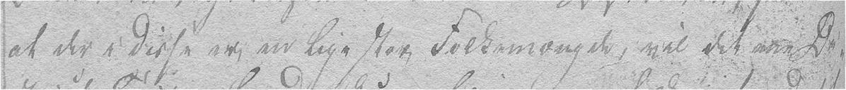at der i disse er en lige stor Folkemængde, vil det ene Di-
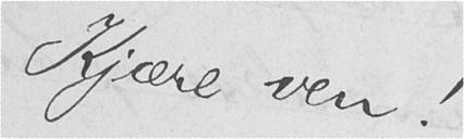Kjære ven!
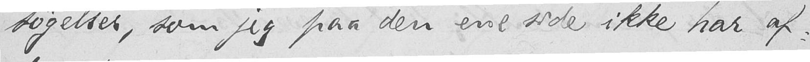søgelser, som jeg paa den ene side ikke har af-
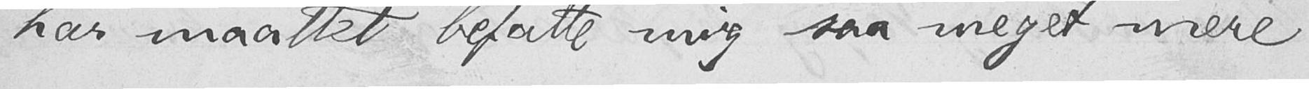har maattet befatte mig saa meget mere
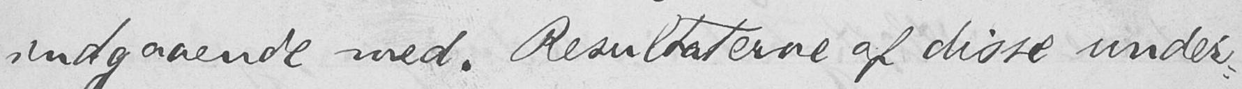indgaaende med. Resultaterne af disse under-
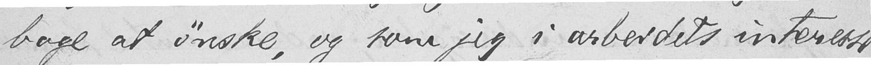bage at ønske, og som jeg i arbeidets interesse
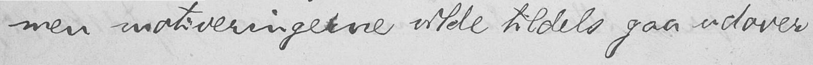men motiveringerne vilde tildels gaa udover
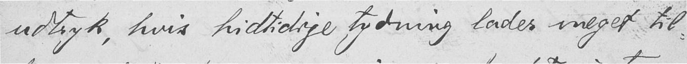udtryk, hvis hidtidige tydning lader meget til-
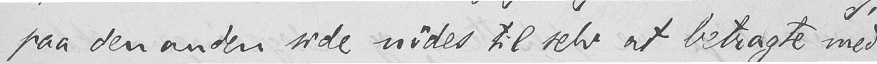paa den anden side nødes til selv at betragte med
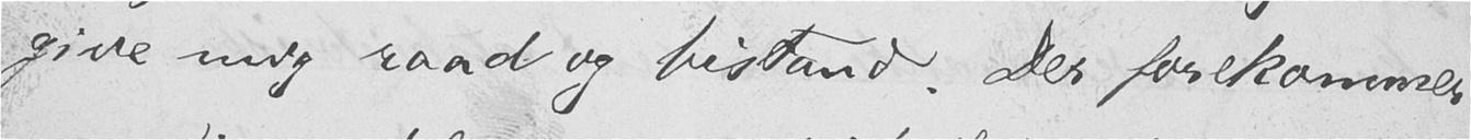give mig raad og bistand. Der forekommer
